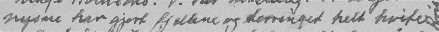nysne har gjort fjellene og terrenget helt hvite
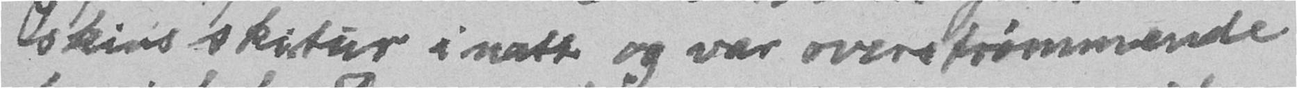skins skitur i natt og var overstrømmende
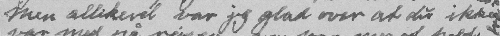men allikevel var jeg glad over at du ikke
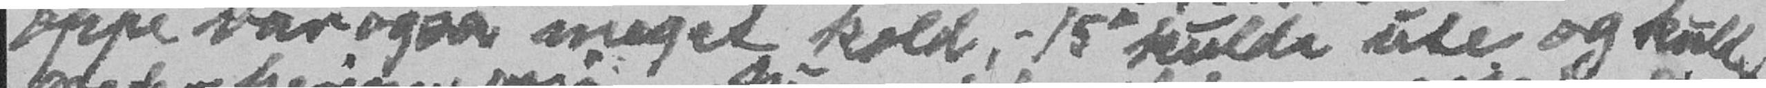oppe var også meget kold, - 15 kulde ute og kulde-
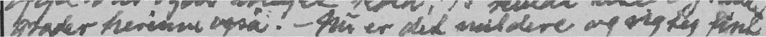grader herinne også! - Nu er det mildere og rigtig fint.
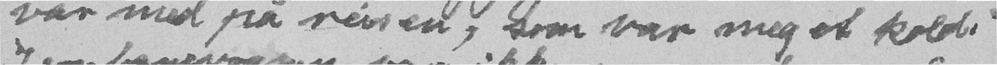var med på reisen, som var meget kold.
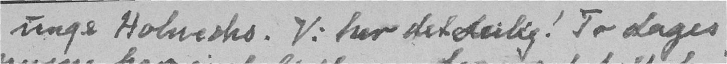unge Holnechs. Vi har det deilig! To dages
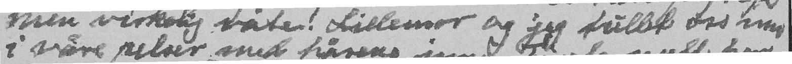men virkelig våte! Lillemor og jeg tullet oss inn
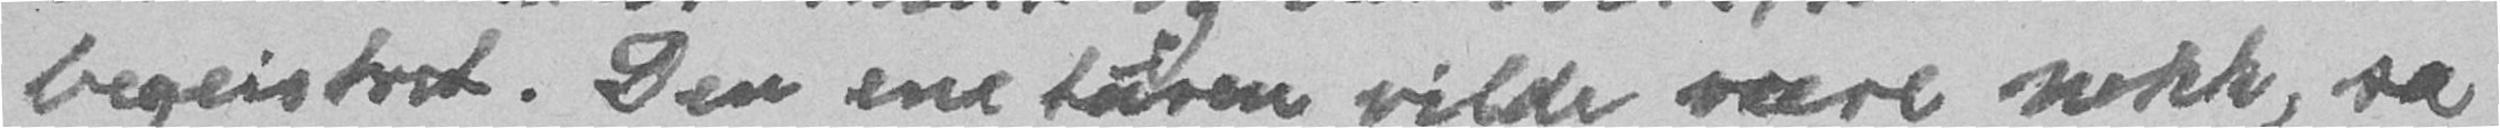begeistret. Den ene turen vilde være nokk, sa
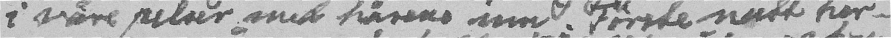i være pelser med hårene inn. Første natt her-
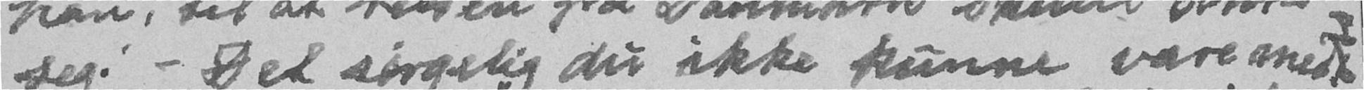seg! - Det sørgelig du ikke kunne være med
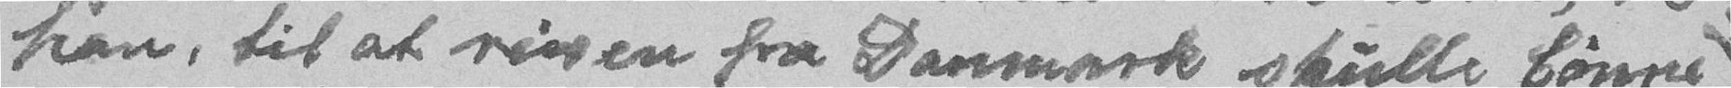han, til at reisen fra Danmark skulle lønne
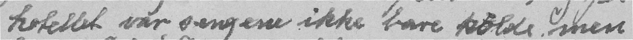hotellet var sengene ikke bare kolde men
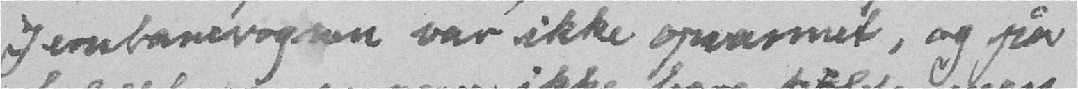Jernbanevognen var ikke opvarmet, og på
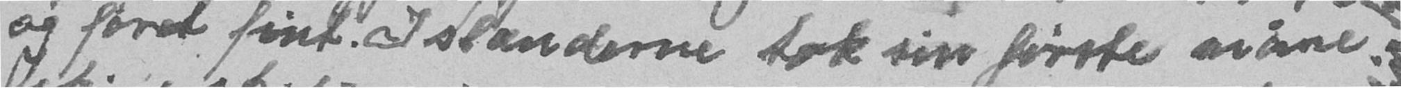og føret fint. Islænderne tok sin første måne-
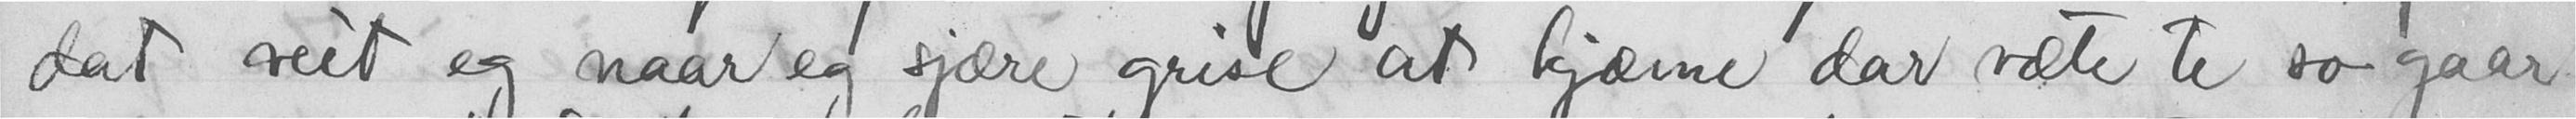dat veit eg naar eg sjære grise at kjæme dar væte te so gaar
